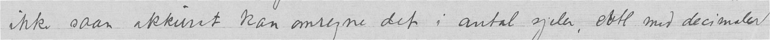ikke saan akkurat kan omregne det i antal sjeler, satt med decimaler
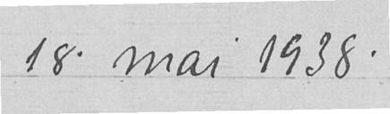18. mai 1938.
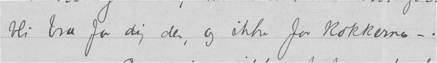bli bra for dig der, og ikke for kokkerne –.
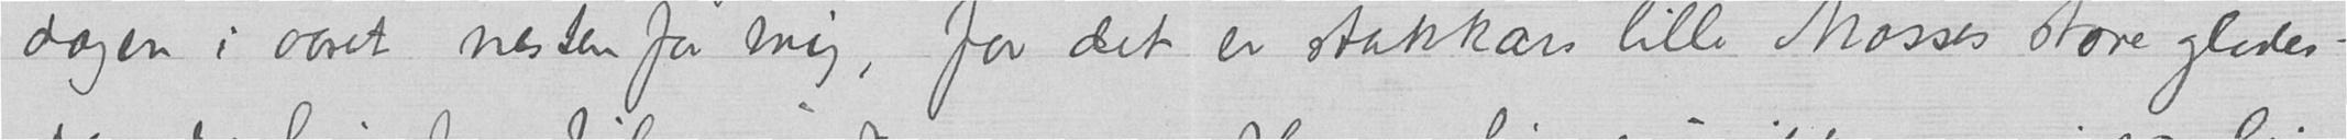dagen i aaret nesten for mig, for det er stakkars lille Mosses store gledes-
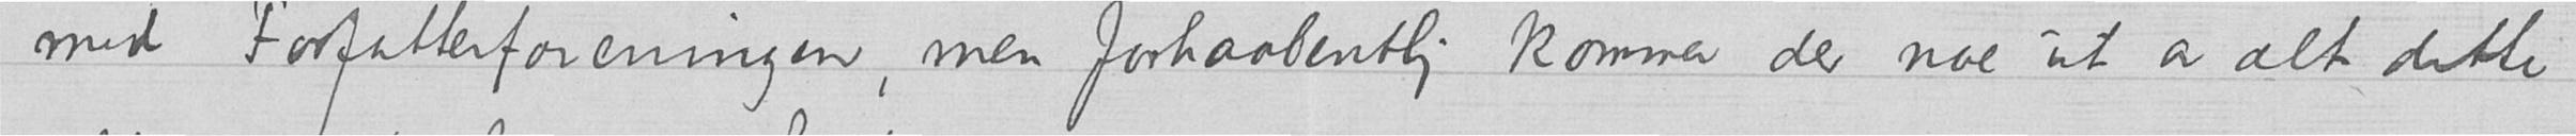med Forfatterforeningen, men forhaabentlig kommer der noe ut av alt dette
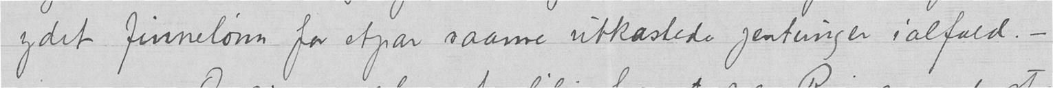ydet finnelønn for etpar saanne utkastede jentunger ialfald. –
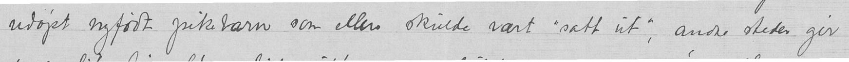udøpt nyfødt pikebarn som ellers skulde vært "satt ut», andre steder gir
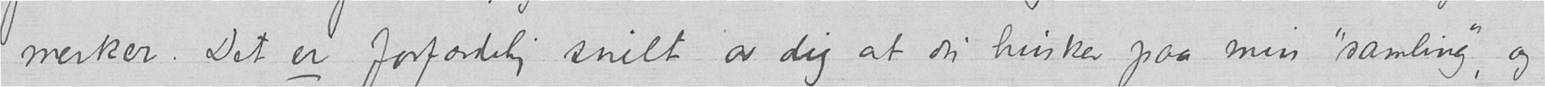merker. Det er forfærdelig snilt av dig at du husker paa min "samling", og
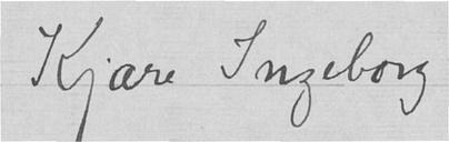Kjære Ingeborg
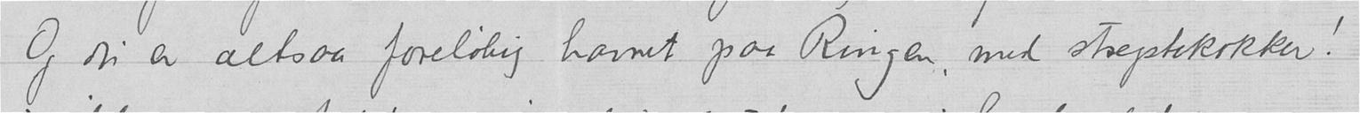Og du er altsaa foreløbig havnet paa Ringen, med streptokokker!
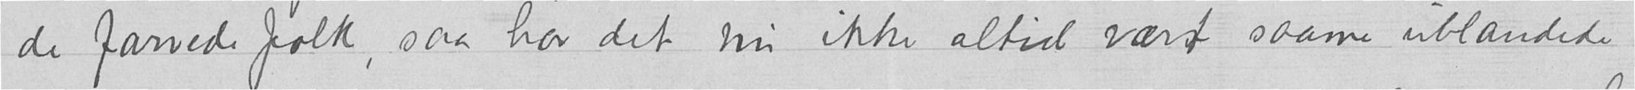de farvede folk, saa har det nu ikke altid vært saane ublandede
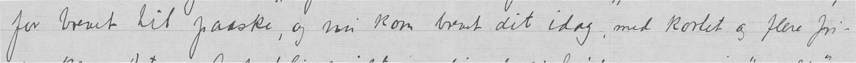for brevet til paaske, og nu kom brevet dit idag, med kortet og flere fri-
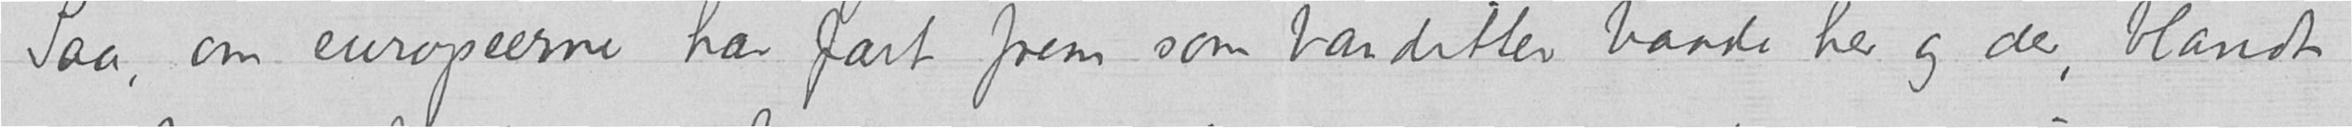Saa, om europeerne har fart frem som banditter baade her og der, blandt
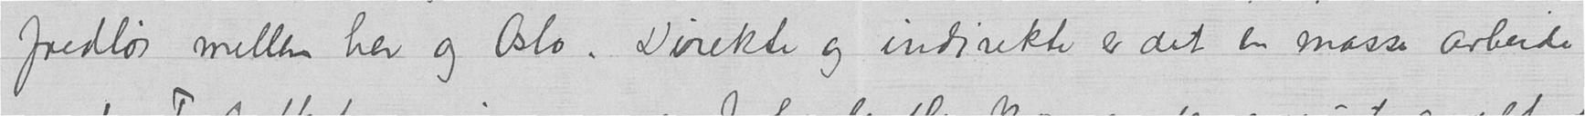fredløs mellem her og Oslo. Direkte og indirekte er det en masse arbeide
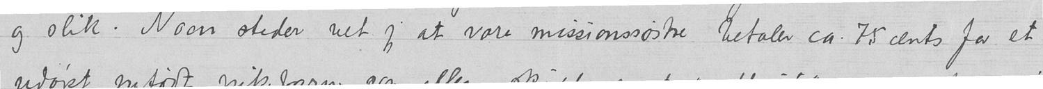og slik. Noen steder vet jeg at vore missionssøstre betaler ca. 75 cents for et
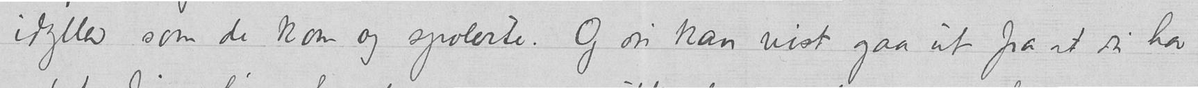idyller som de kom og spolerte. Og du kan vist gaa ut fra at du har
